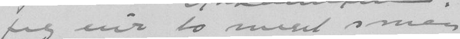Jeg eier to meget smaa
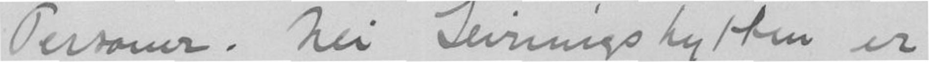Personer. Nei Leirungshytten er
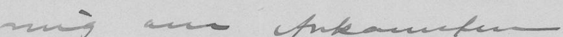mig om Ankomsten.
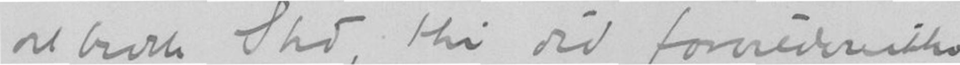det bedste Sted, thi did forvilder
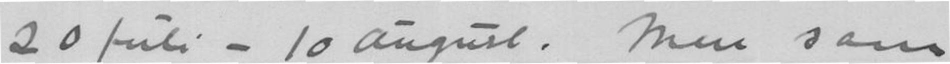20 Juli - 10 August. Men som
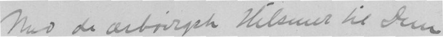Med de ærbødigst Hilsener til Dem
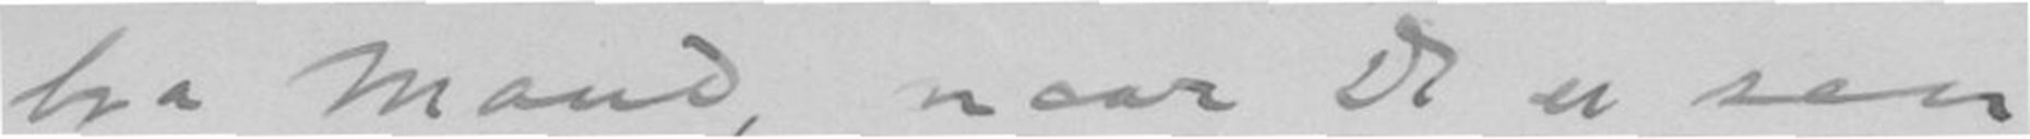bra Mand, naar De er saa
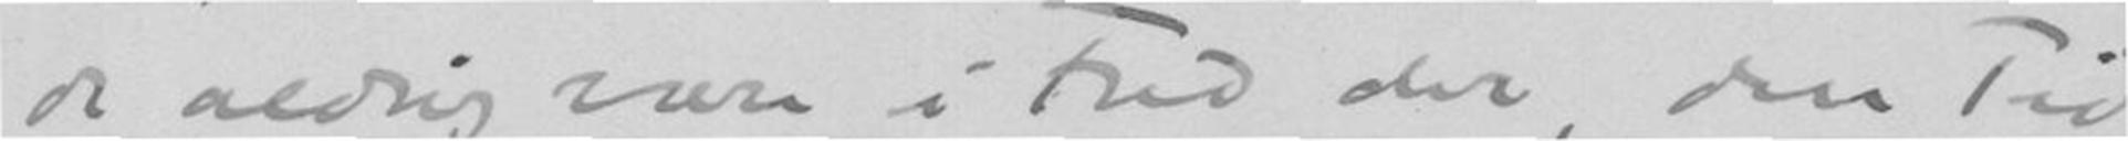de aldrig være i Fred der, den Tid
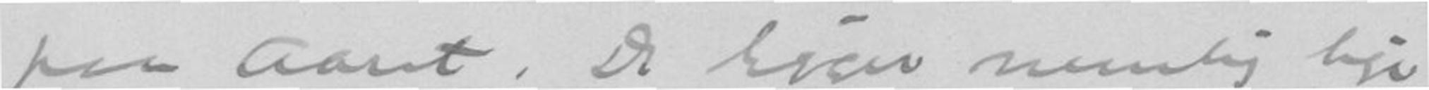paa Aaret. De ligger nemlig lige
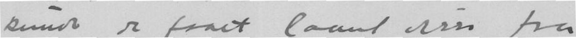kunde de faaet laant disse fra
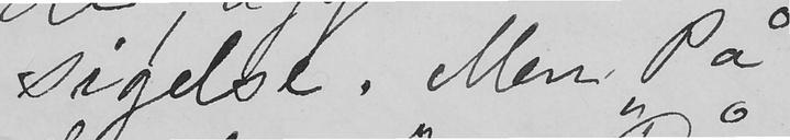sigelse. Men "På
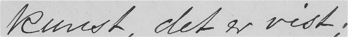kunst, det er vist;
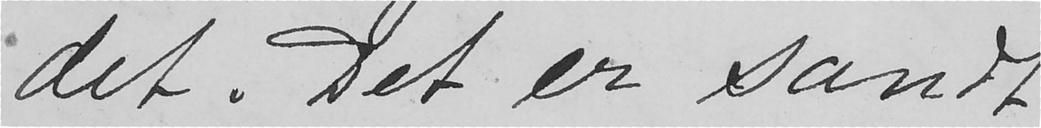det. Det er sandt
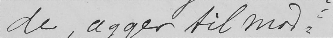de, ægger til mod-
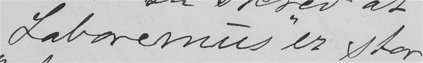"Laboremus" er stor
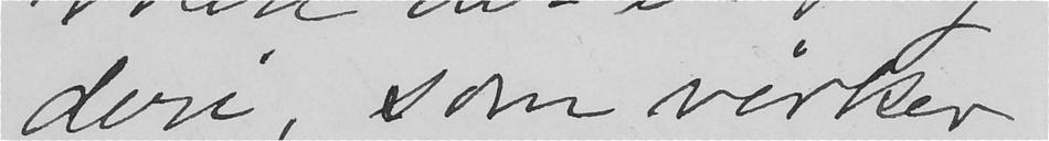deri som virker
